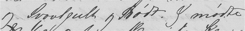og Svovlgult og Rødt. Jeg mødte
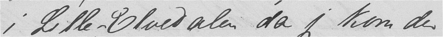i Lille-Elvedaten, da jeg kom der
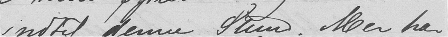indtil denne Stund. Mer har
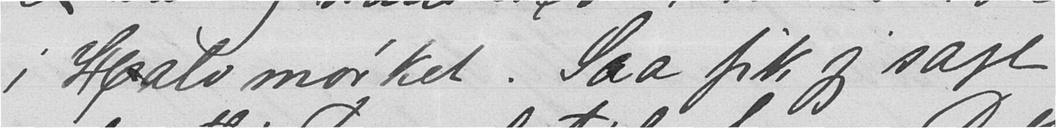i Halv mørket. Saa fik jeg sagt
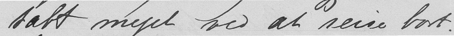tabt meget ved at reise bort,
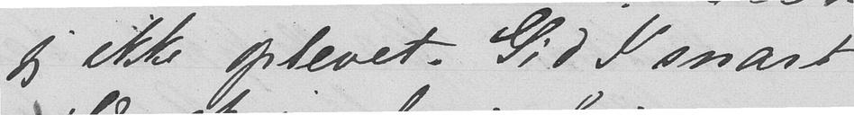jeg ikke oplevet. Gid I snart
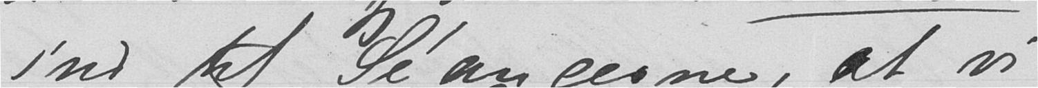ind til le'ancerne, at vi
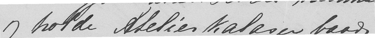og holde Atelierkalaser baade
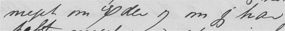meget om Eder og om jeg har
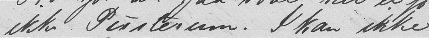ikke Pusterum. I kan ikke
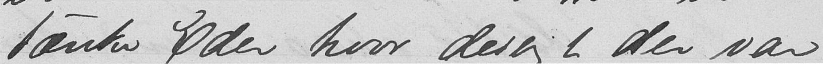tænke Eder hvor deiligt der var
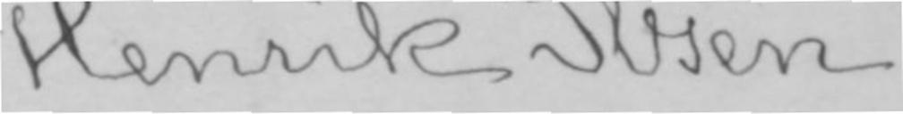Henrik Ibsen.
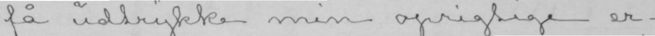få udtrykke min oprigtige er-
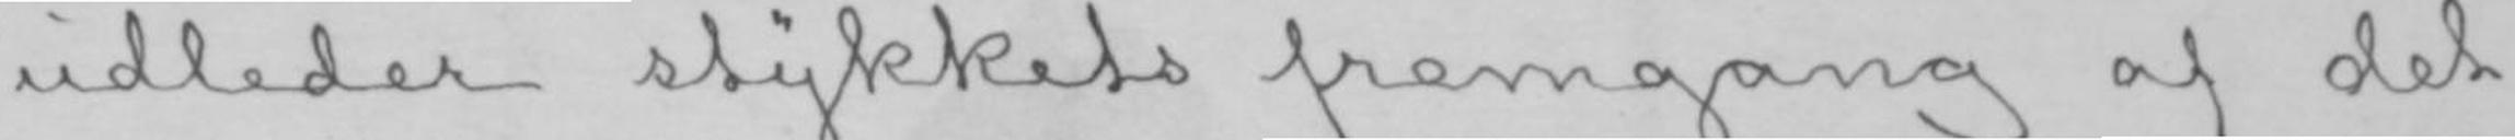udleder stykkets fremgang af det
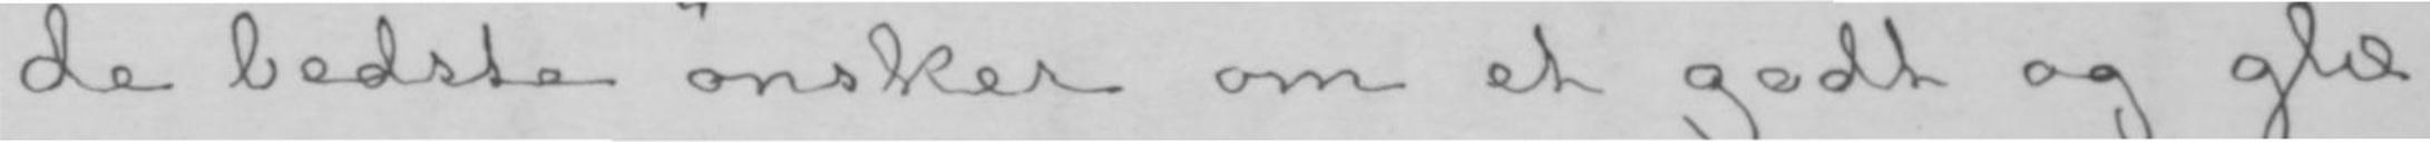de bedste ønsker om et godt og glæ-
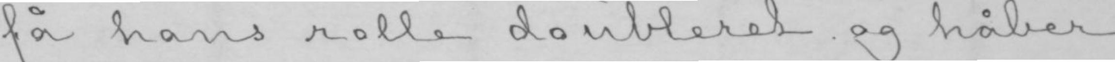få hans rolle doubleret og håber
